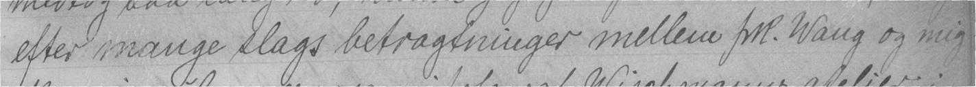efter mange slags betragtninger mellem frk. Wang og mig
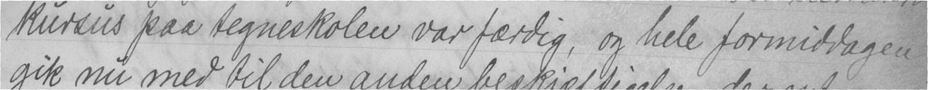kursus paa tegneskolen var færdig, og hele formiddagen
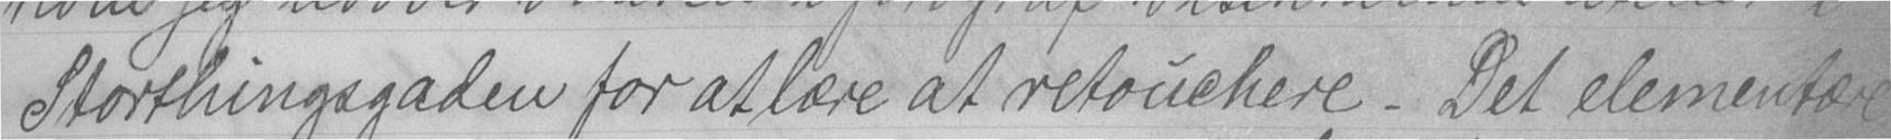Storthingsgaden for at lære at retouchere. Det elementære
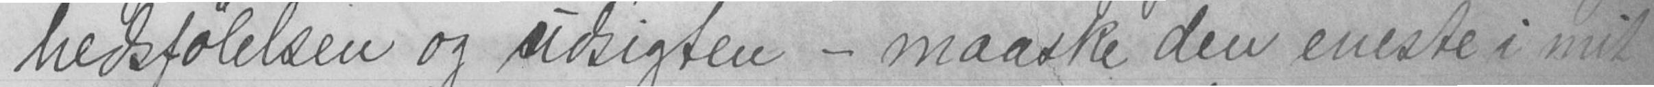hedsfølelsen og udsigten - maaske den eneste i mit
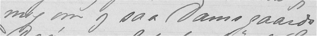mig om og saa Damsgaards
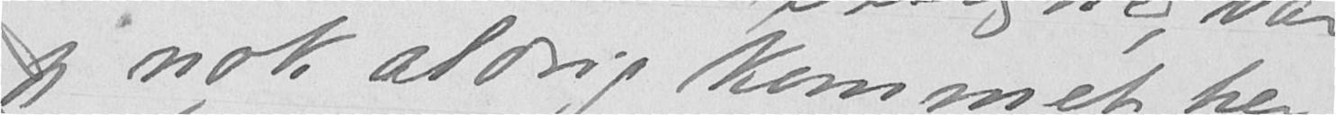jeg nok aldrig kommet her,
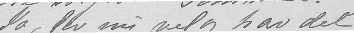Ja, lev nu vel og hav det
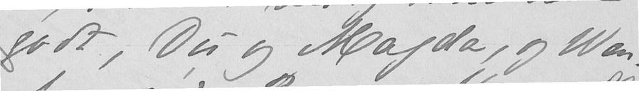godt, Du og Magda, og Wen-
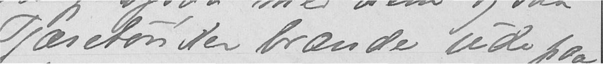Tjæretønder brænde ude paa
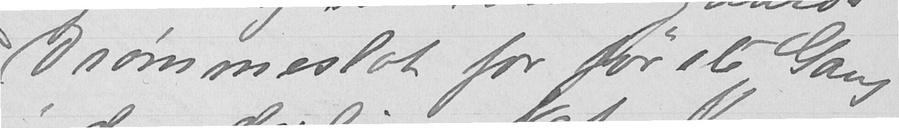Drømmeslot for første Gang
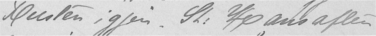Rusten igjen. St. Hansaften
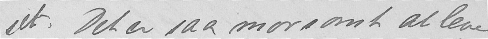set. Det er saa morsomt at leve
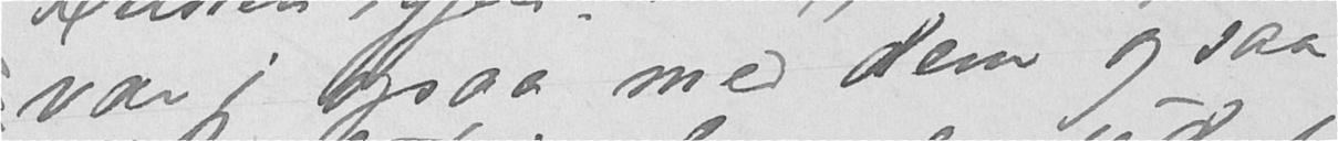var jeg ogsaa med dem og saa
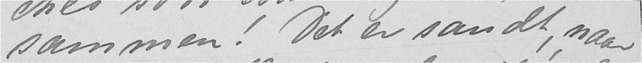sammen! Det er sandt, naar
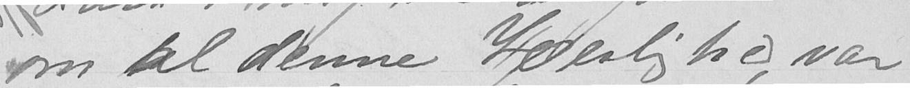om al denne Herlighed, var
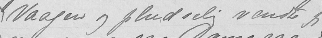Vaagen og pludselig vandt jeg
